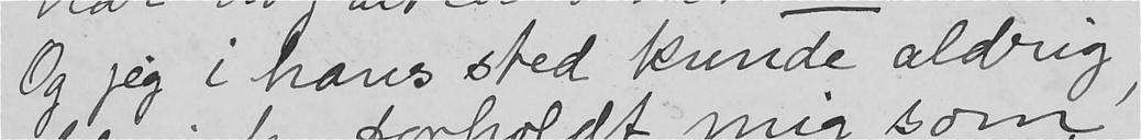Og jeg i hans sted kunde aldrig,
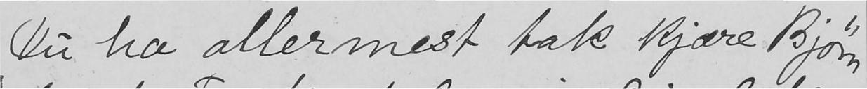Du ha allermest tak kjære Bjørn-
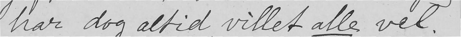har dog altid villet alle vel.
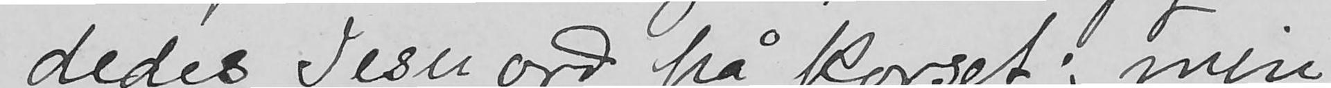dedes Jesu ord på korset: "min
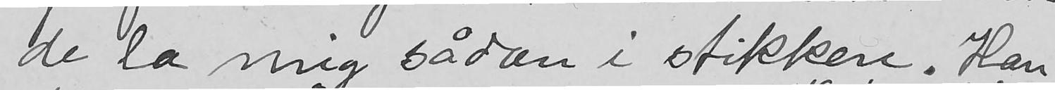de la mig såden i stikken. Han
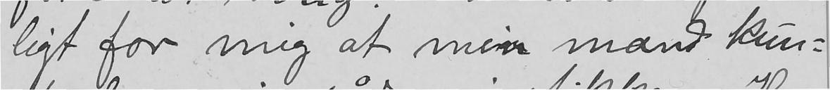ligt for mig at min mand kun-
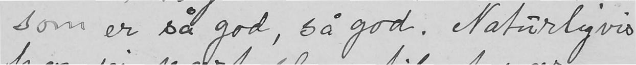som er så god, så god. Naturligvis
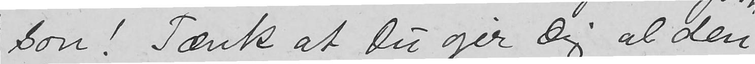son! Tænk at Du gir Dig al den
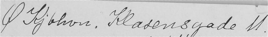Ø Kjbhvn Klasensgade 11.
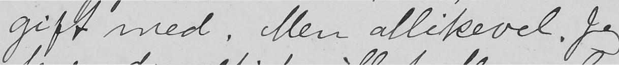gift med. Men allikevel. Jeg
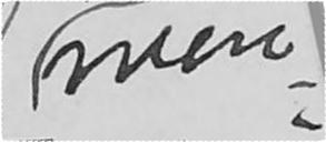men-
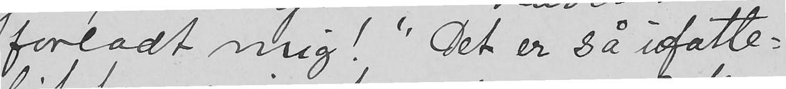forladt mig!" Det er så ufatte-
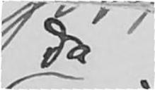da
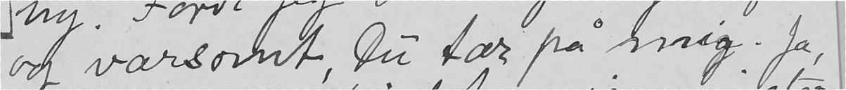og varsomt, Du tar på mig. Ja,
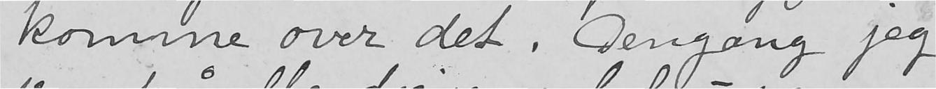komme over det. Dengang jeg
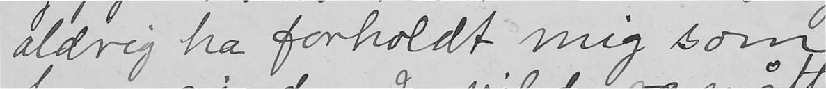aldrig ha forholdt mig som
